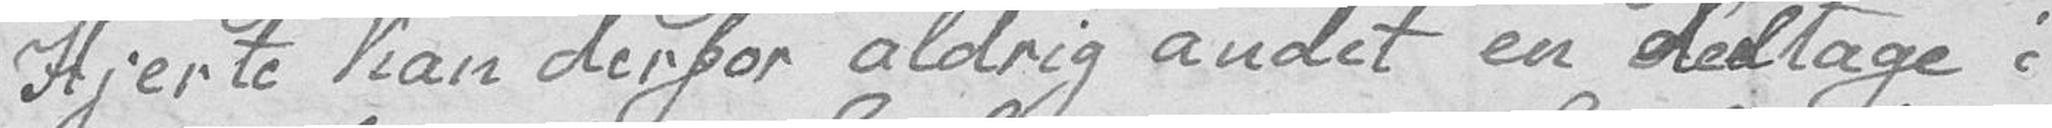Hjerte kan derfor aldrig andet en deltage i
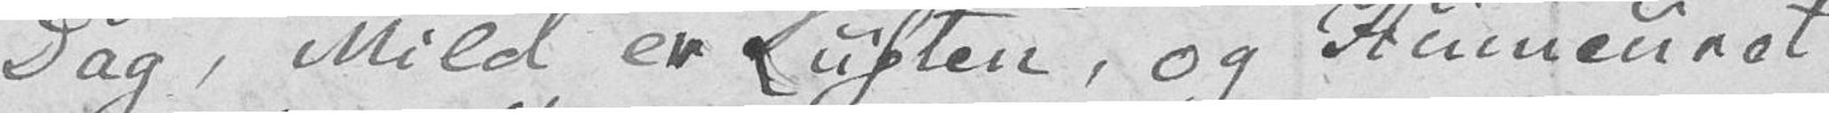Dag, Mild er Luften, og Humeuret
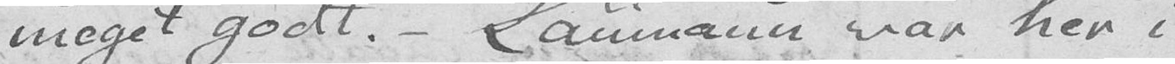meget godt. – Laumann var her i
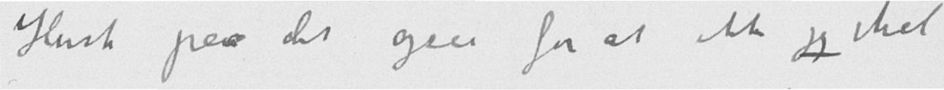Husk paa det ogsaa for at ikke jeg skal
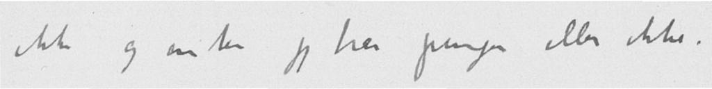ikke og enten jeg har penger eller ikke.
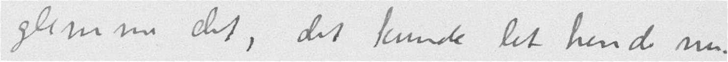glemme det, det kunde let hende nu.
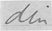din
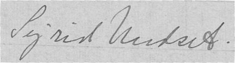Sigrid Undset.
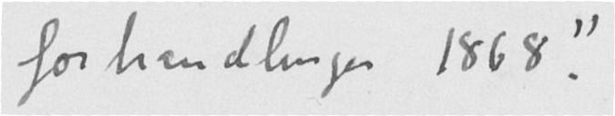forhandlinger 1868."
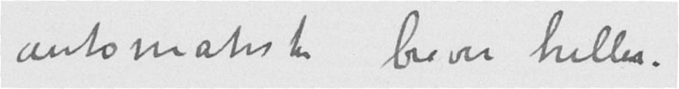automatiske brever heller.
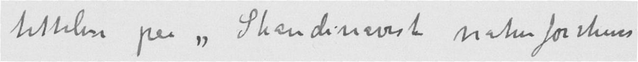tittelen paa "Skandinaviske naturforskeres
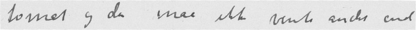tomat og du maa ikke vente andet end
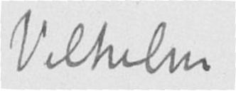Vilhelm
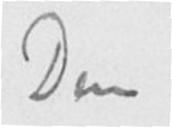Din
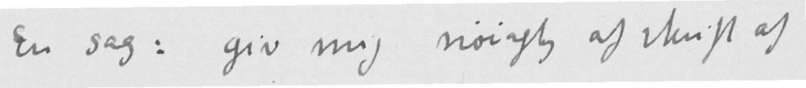En sag: giv mig nøiagtig afskrift af
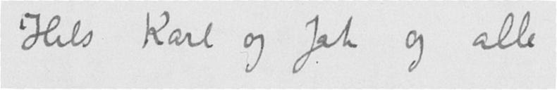Hils Karl og Jak og alle
